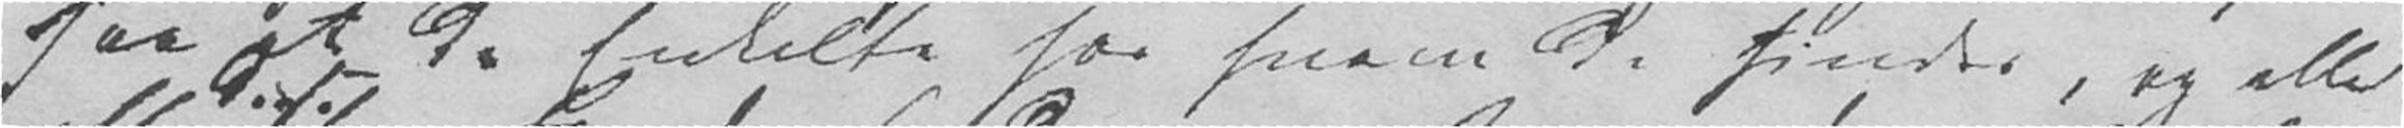saa at de Enkelte for hvem de findes, og alle
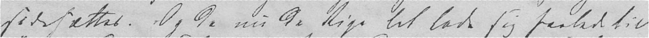sidesættes. Og da nu de Rige let lade sig forlede til
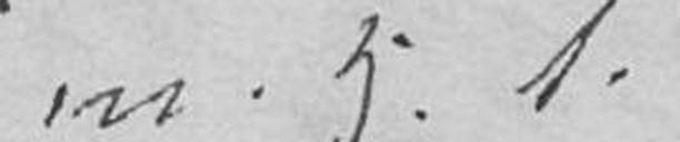m. H. t.
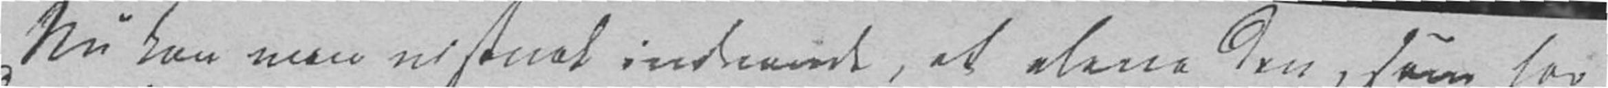Nu kan man vistnok indvende, at alene den, som har
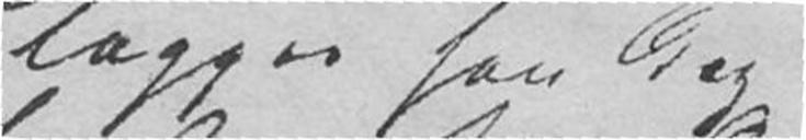lægger han dog
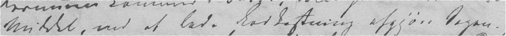Middel, end at lade Lodkastning afgjøre Sagen.
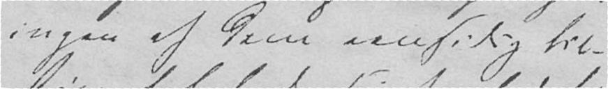ingen af dem eensidig til-
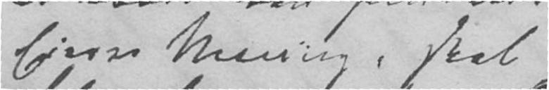Eieres Mening, skal
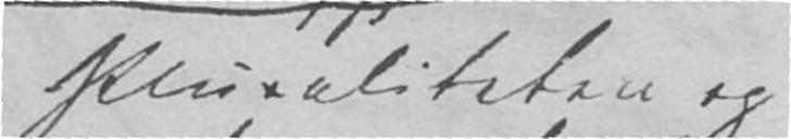Pluraliteten og
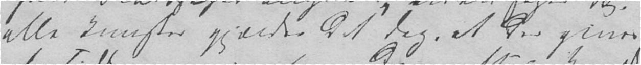alle Kunster gjælder det dog, at der gives
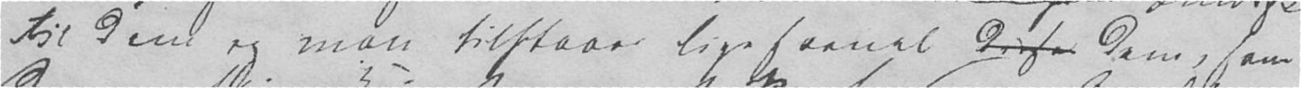til dem og man tilstaaer lige saavel disse dem, som
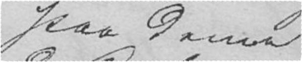Paa denne
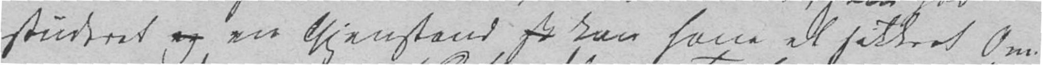studeret og en Gjenstand sk kan have al sikkret Om-
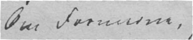Om Formerne,
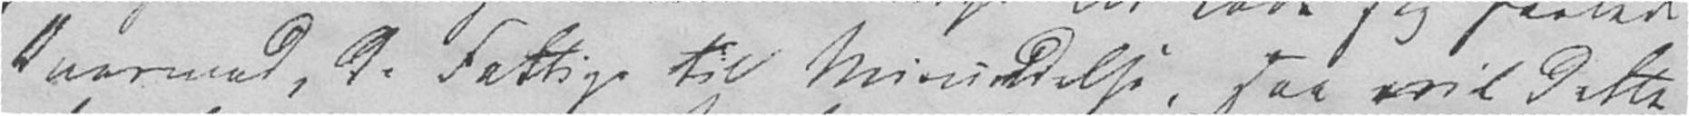Overmod, de Fattige til Misundelse, saa vil dette
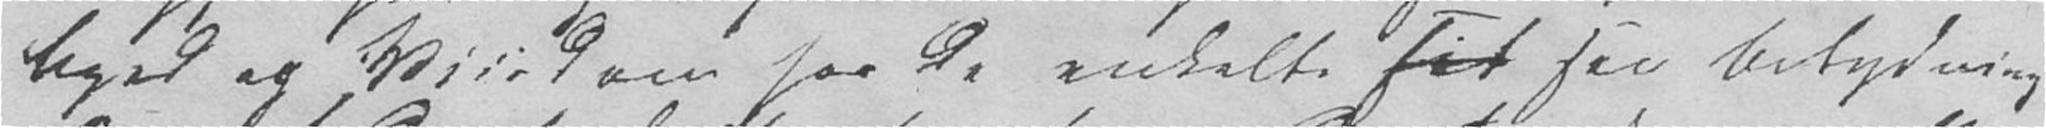lighed og Viisdom hos de enkelte sit sin Betydning
